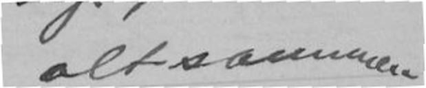Altsammen
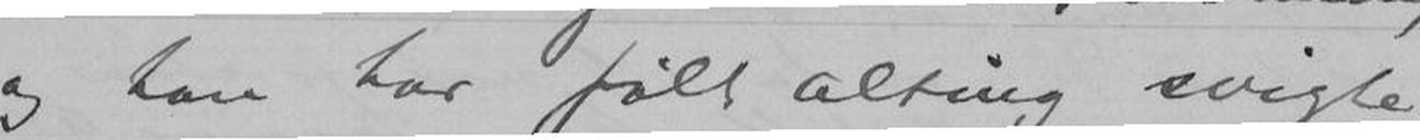og han har følt alting svigte
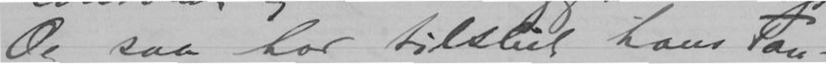Og saa har tilslut hans Tan-
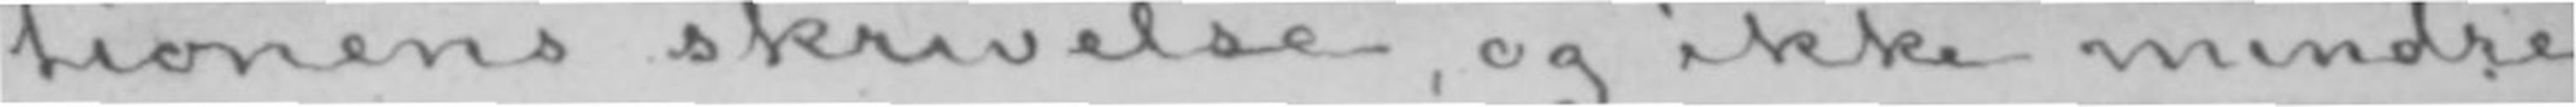direktionens skrivelse, og ikke mindre
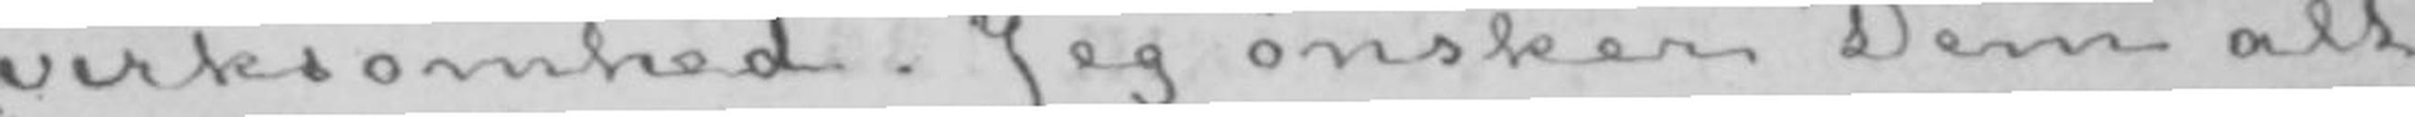virksomhed. Jeg ønsker Dem alt
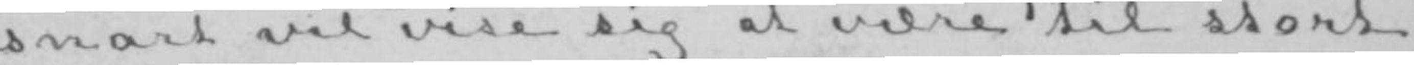snart vil vise sig at være til stort
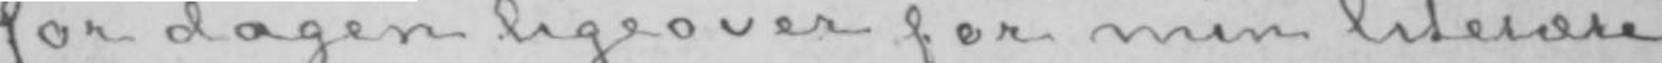for dagen ligeover for min literære
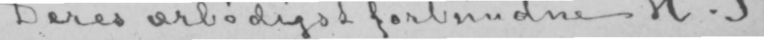Deres ærbødigst forbundne H. I.
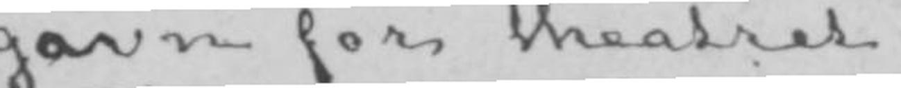gavn for theatret.
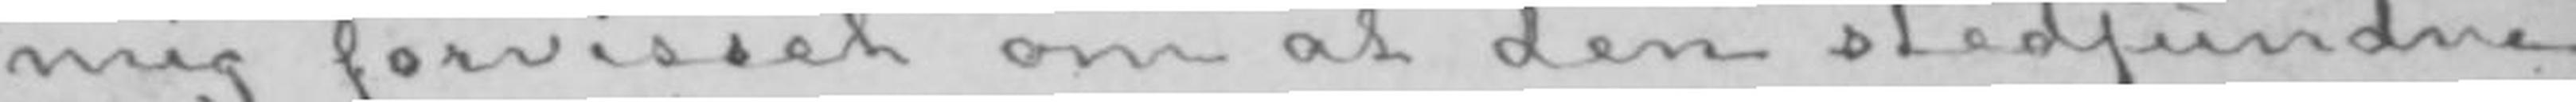mig forvisset om at den stedfundne
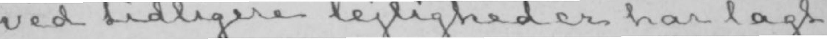ved tidligere lejligheder har lagt
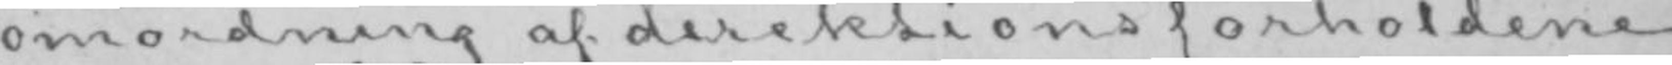omordning af direktionsforholdene
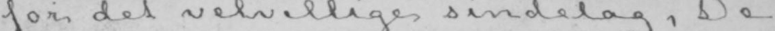for det velvillige sindelag, De
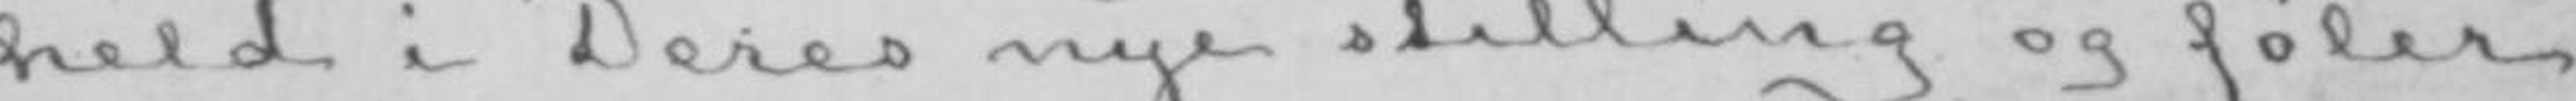held i Deres nye stilling og føler
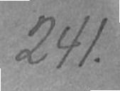241.
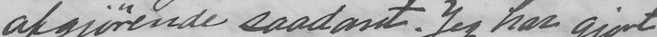afgjørende saadant. Jeg har gjort
